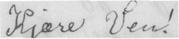Kjære Ven!
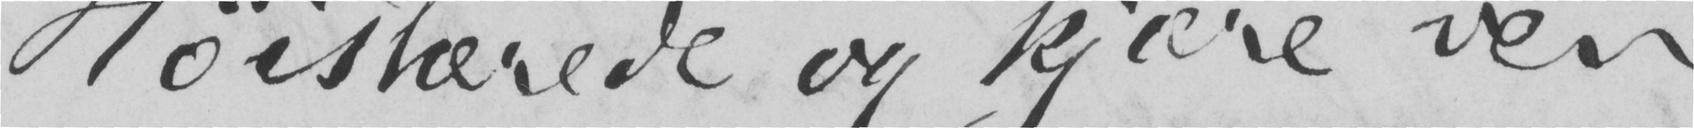Høistærede og kjære ven!
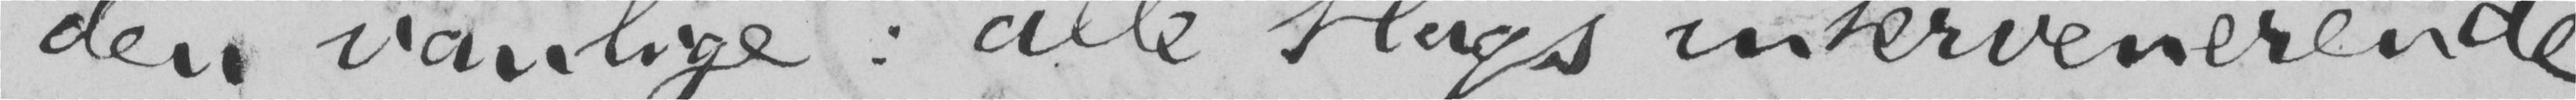den vanlige: alle slags intervenerende
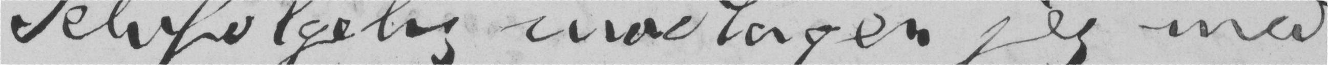Selvfølgelig modtager jeg med
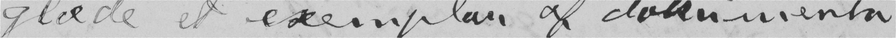glæde et exemplar af «dokumenta-
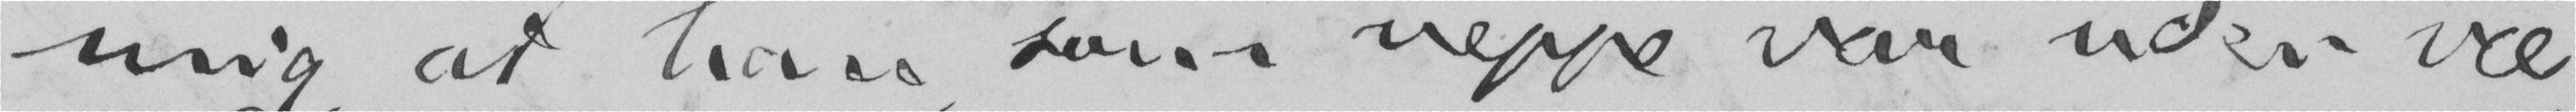mig, at han, som neppe var uden væ-
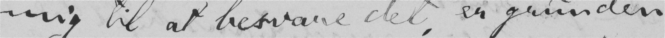mig til at besvare det, er grunden
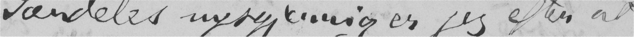Særdeles nysgjerrig er jeg efter at
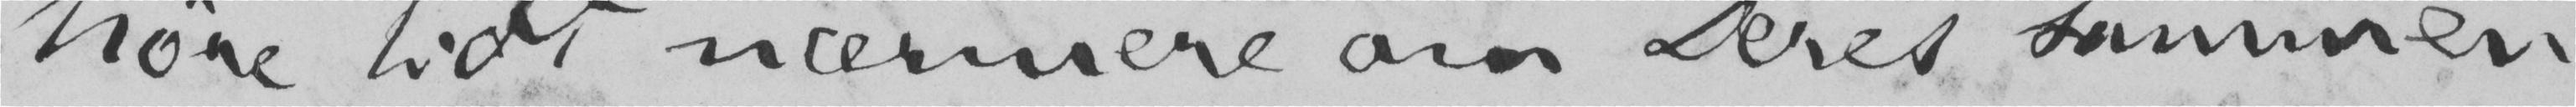høre lidt nærmere om Deres sammen-
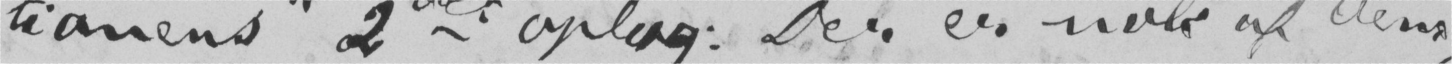tionens» 2det oplag: Der er nok af dem,
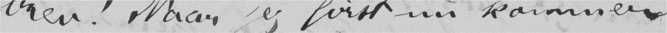brev! Naar jeg først nu kommer
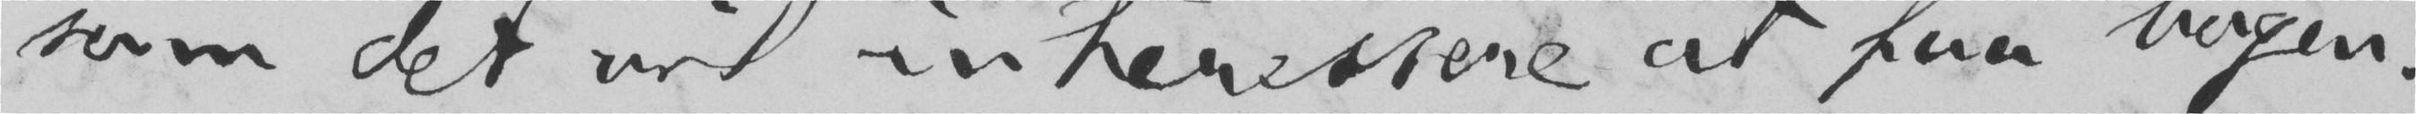som det vil interessere at faa bogen!
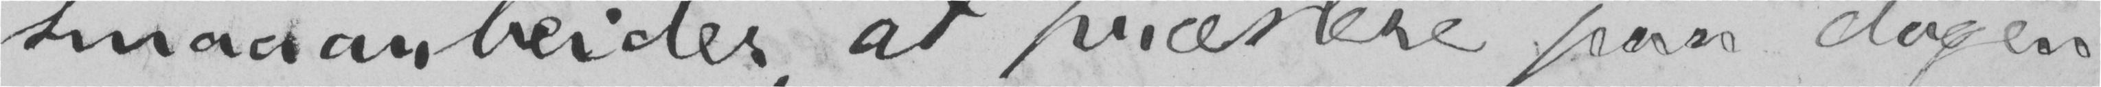smaaarbeider, at præstere paa dagen.
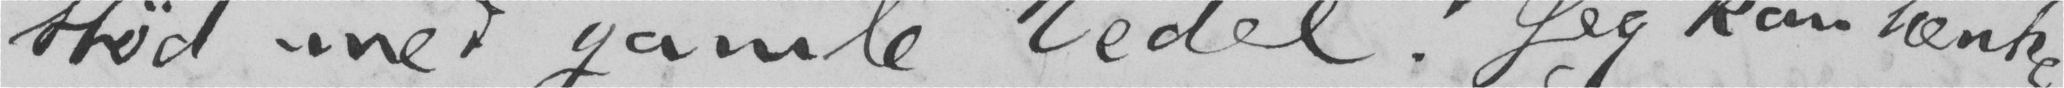stød med gamle Vedel! Jeg kan tænke
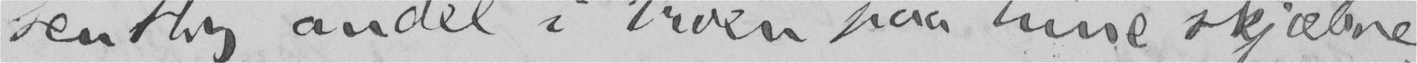sentlig andel i troen paa hine skjæbne-
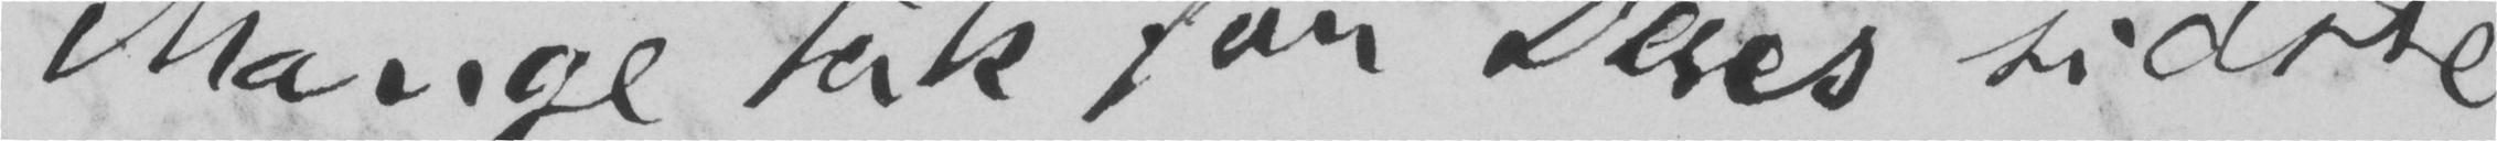Mange tak for Deres sidste
